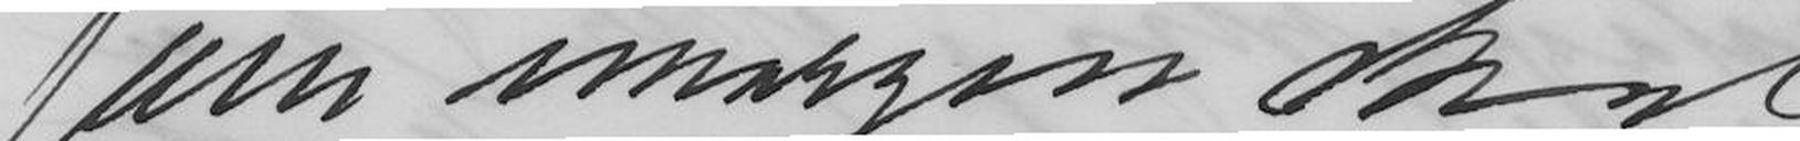som imorgen skal
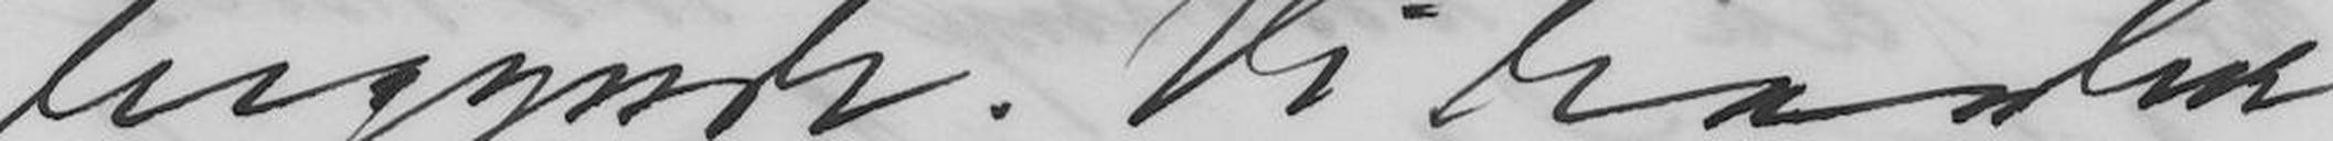begynde. Vi haaber
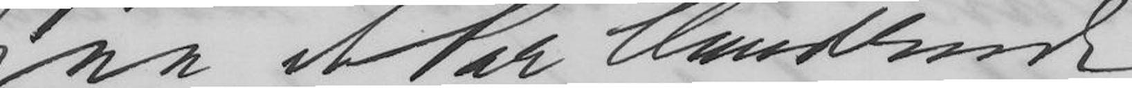paa et Par Hundrede
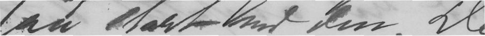saa stort ved den. De
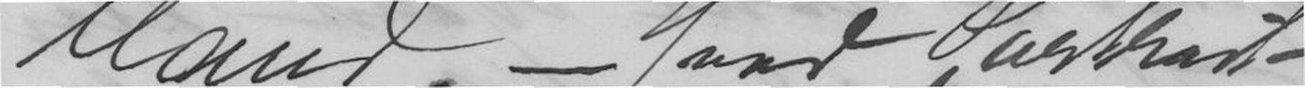Mand. - Hvad Portrait
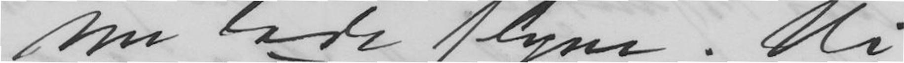nu lade flyve. Vi
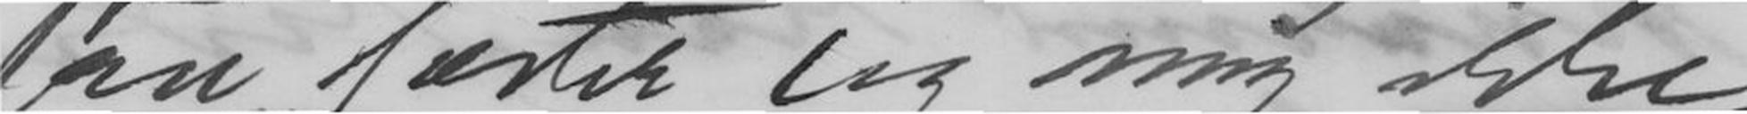saa fæster jeg mig ikke
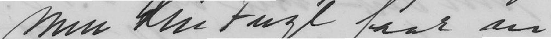men den Fugl faar vi
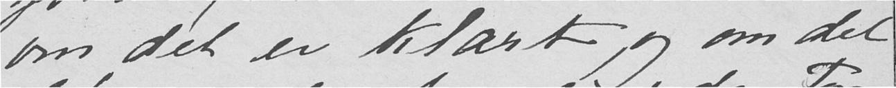om det er klart, og om det
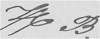H. B.
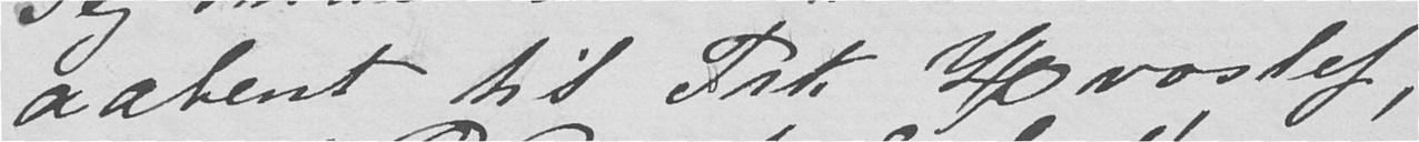aabent til Frk Hvoslef,
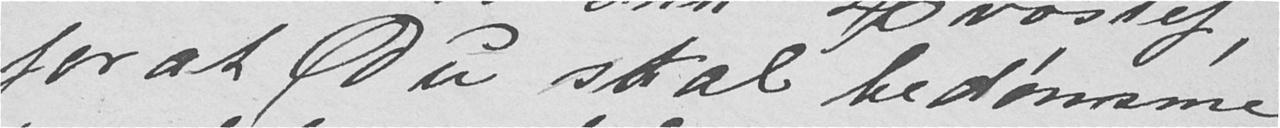for at Du skal bedømme
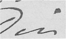Din
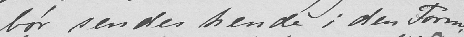bør sendes hende i den Form.
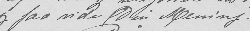og faa vide Din Mening.
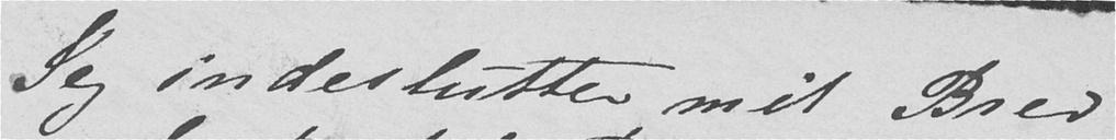Jeg indeslutter mit Brev
